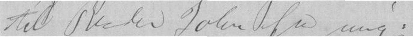til Broder John fra mig.
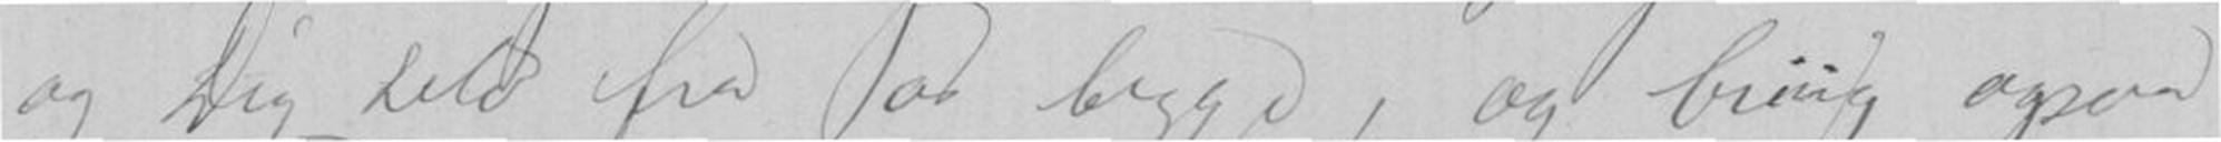og Dig selv fra os begge, og bring ogsaa
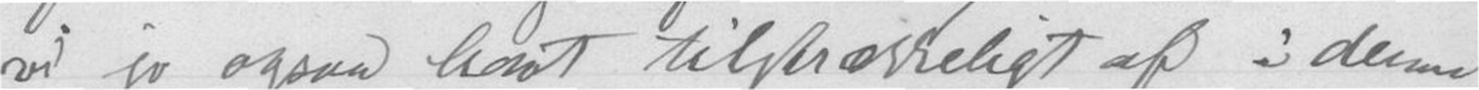vi jo ogsaa havt tilstrækkeligt af i denne
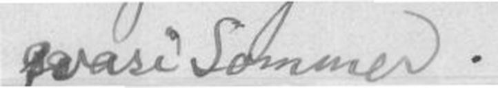quasi Sommer.
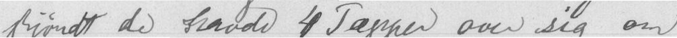skjøndt de havde 4 Tæpper over sig om
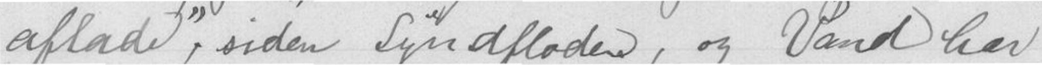aflade, siden Syndfloden, og Vand har
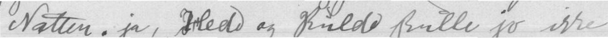Natten; ja, Hede og Kulde skulle jo ikke
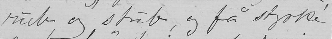rub og stub, og få styrke
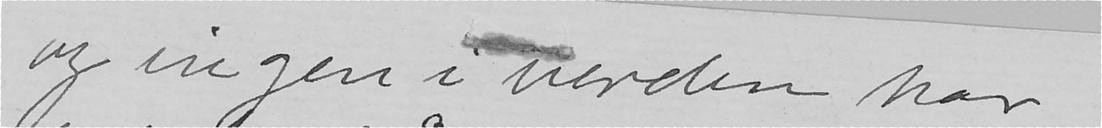og ingen i verden har
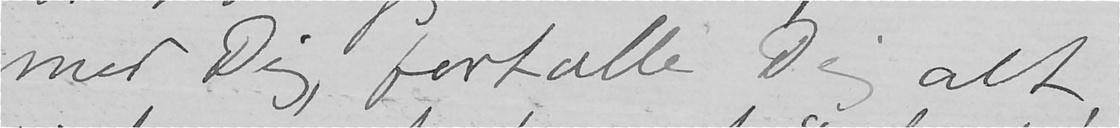med Dig, fortælle Dig alt,
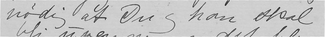nødig at Du og han skal
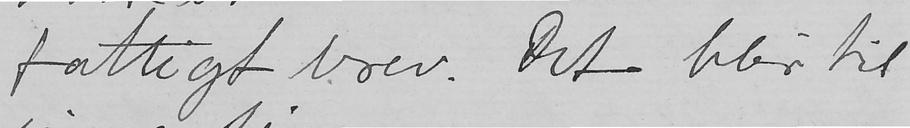fattigt brev. Det blir til
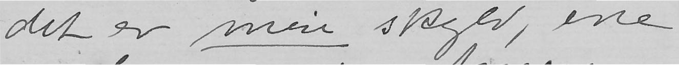det er min skyld, ene
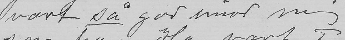vært så god imod mig
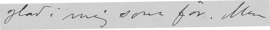glad i mig som før. Men
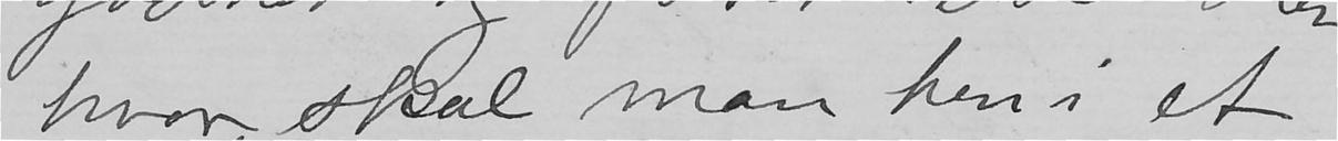hvor skal man hen i et
